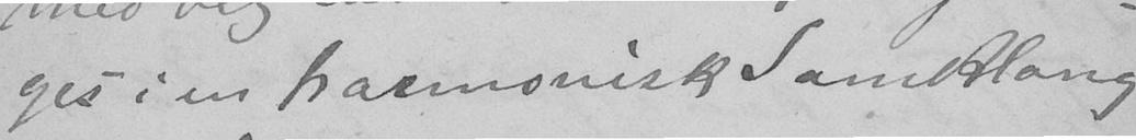ges i en harmonisk Samklang
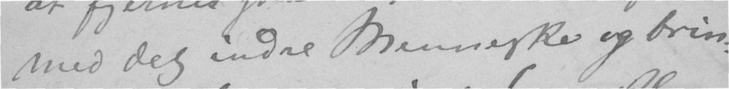med det indre Menneske og brin-
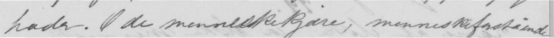hæder. O de menneskekjære, menneskeforstående
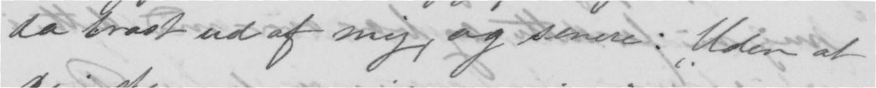da brast ud af mig, og senere: "Uden at
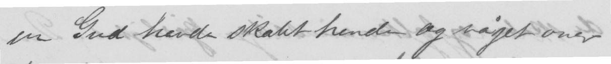en Gud havde skabt hende og våget over
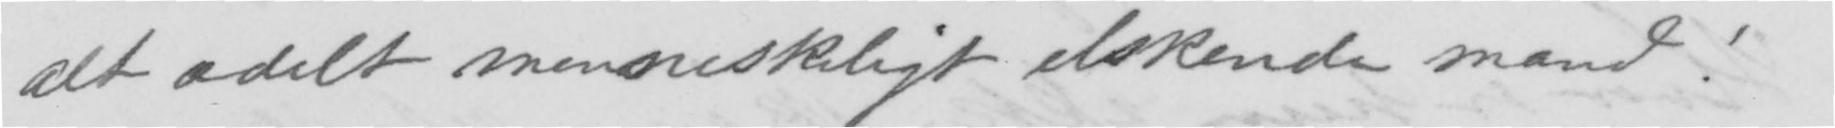alt ædelt menneskeligt elskende mand!
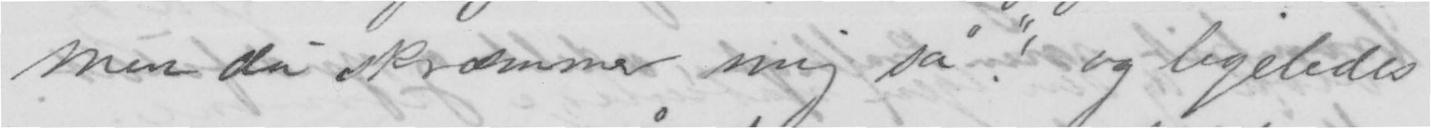men du skræmmer mig så"! og ligeledes
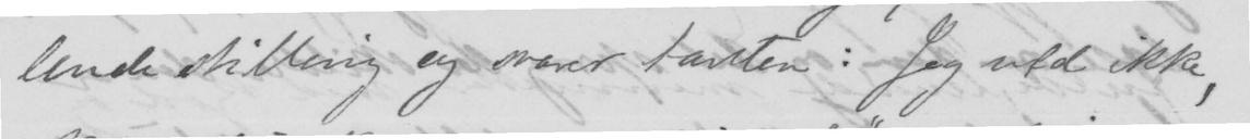lende stilling og svarer tanten: "Jeg vild ikke,
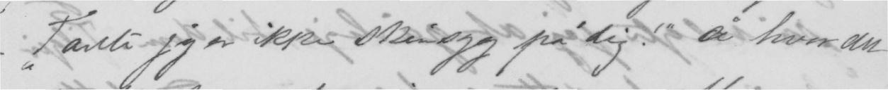"Tante jeg er ikke skinsyg på dig!" å hvor det
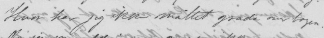Hvor har jeg ikke måttet græde over bogen.
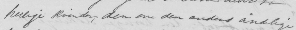herlige kvinder, den ene den andens åndelige
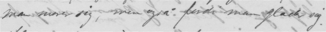man morer sig, men også fordi man glæder sig.
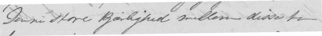Denne store Kjærlighed mellem disse to
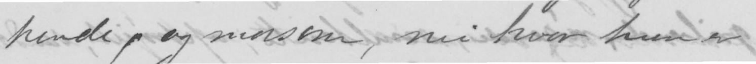hende, og morsom, nei hvor hun er
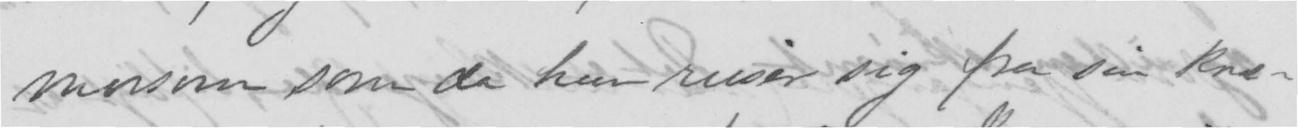morsom som da hun reiser sig fra sin knæ-
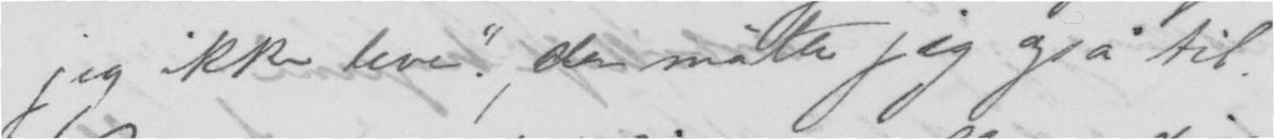jeg ikke leve" da måtte jeg også til.
